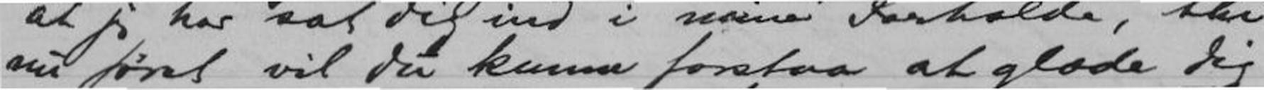nu først vil Du kunne forstaa at glæde Dig
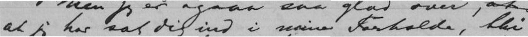at jeg har sat Dig ind i mine Forholde, thi
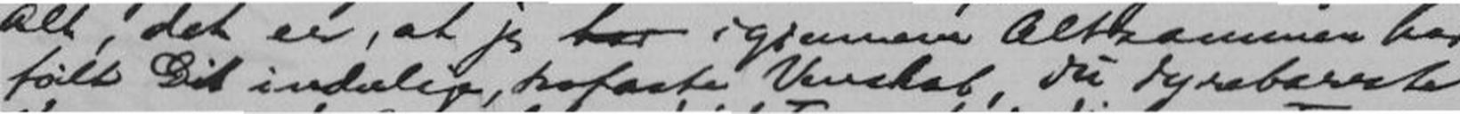følt Dit inderlige, trofaste Venskab, du dyrebareste
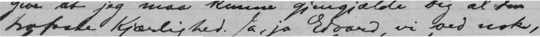trofaste Kjærlighed. Ja, ja Edvard, vi ved nok,
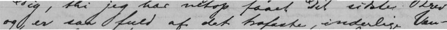og er saa fuld af det trofaste, inderlige Ven-
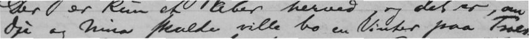Du og Nina skulde ville bo en Vinter paa Trold-
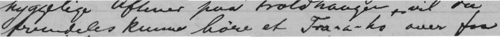fremdeles kunne høre et Tra-a-ho over fra
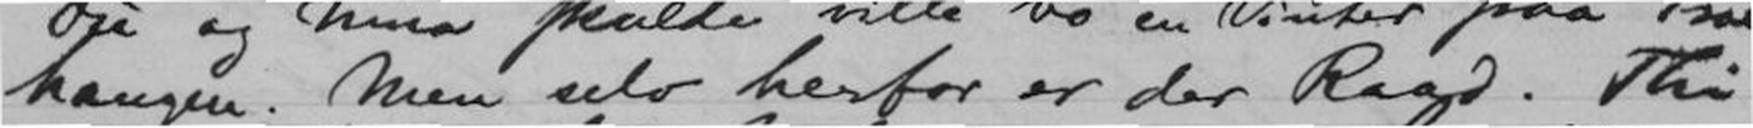haugen. Men selv herfor er det Raad. Thi
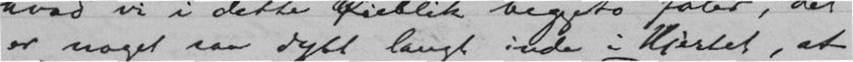er noget saa dybt langt inde i Hjertet, at
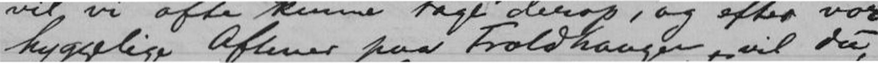hyggelige Aftener paa Troldhaugen vil Du
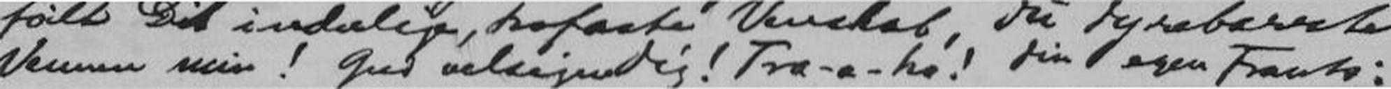Vennen min! Gud velsigne dig! Tra-a-ho! Din egen Frants.
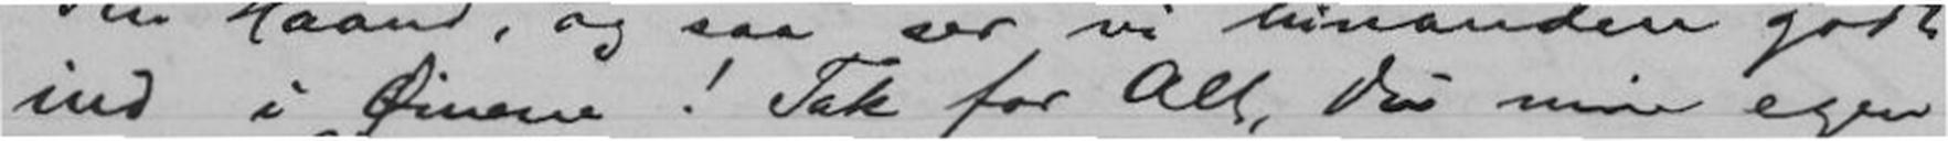ind i Øinene! Tak for Alt, du min egen
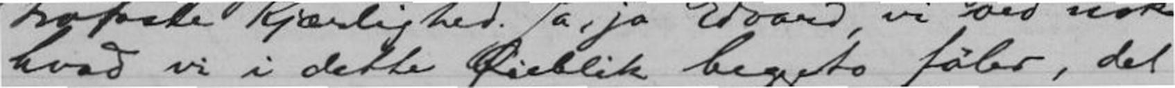hvad vi i dette Øieblik beggeto føler, det
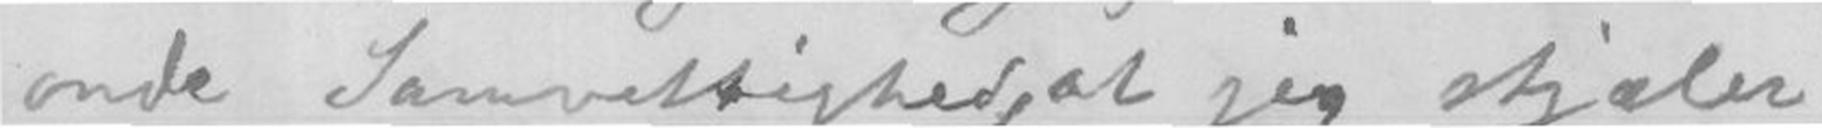onde Samvittighed, at jeg stjæler
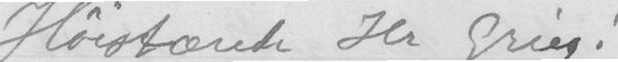Høistærede Hr Grieg!
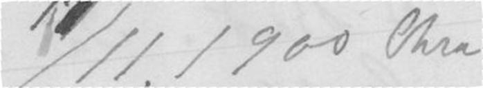17/11.1900 Chra
